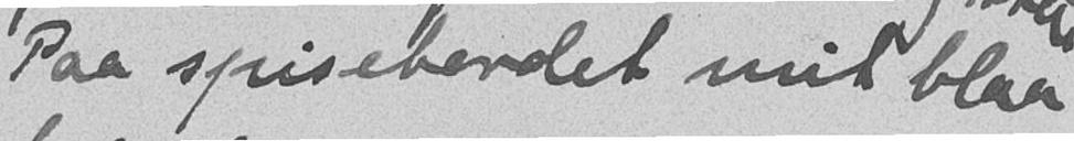Paa spisebordet mit blaa
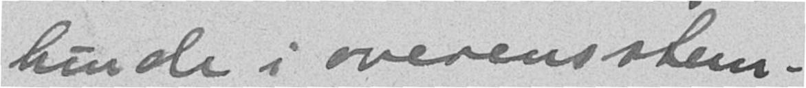lunde i overensstem-
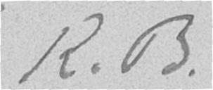K.B.
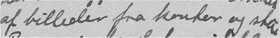af billeder fra kontor og stue.
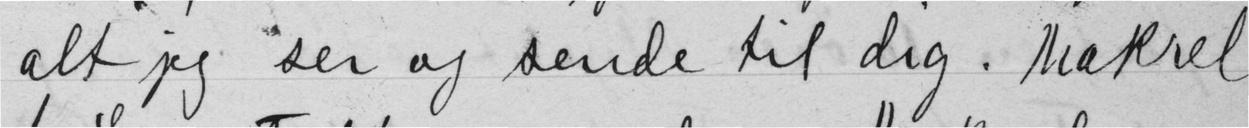alt jeg ser og sende til dig. Makrel
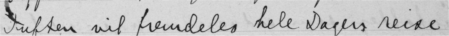Tuften vil fremdeles hele Dagers reise
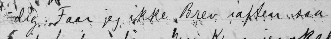dig. Faar jeg ikke Brev iaften saa
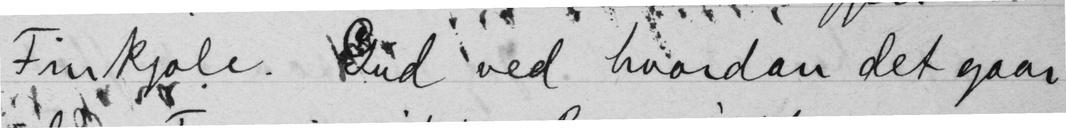Finkjole. Gud ved hvordan det gaar
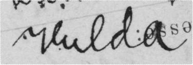Hulda
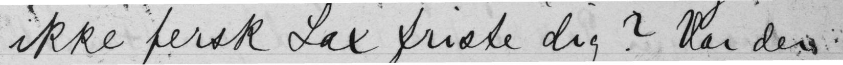ikke fersk Lax friste dig? Har dere
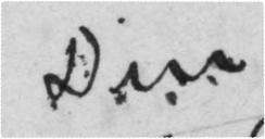Din
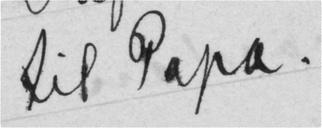til Papa.
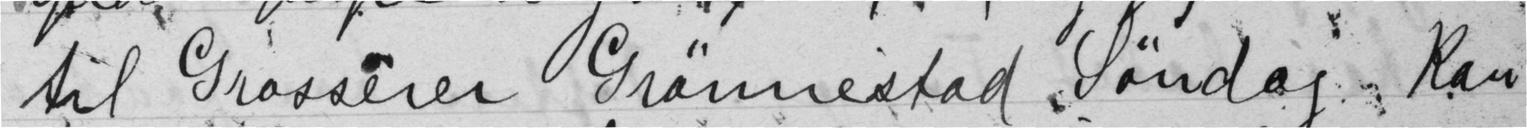til Grosserer Grønnestad Søndag kan
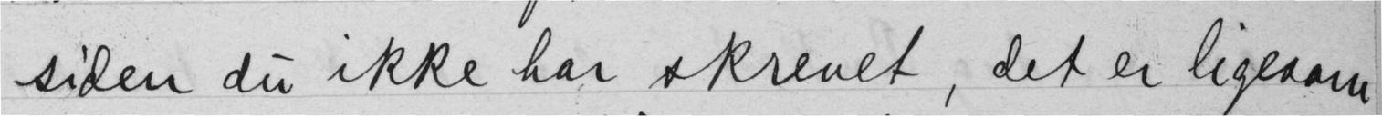siden du ikke har skrevet, det er ligesom
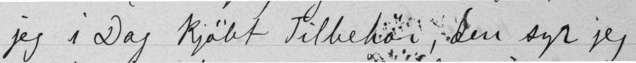jeg i Dag Kjøbt Tilbehør, den syr jeg
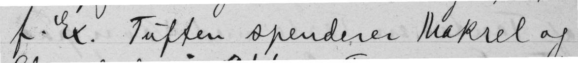f. ex. Tuften spenderer Makrel og
